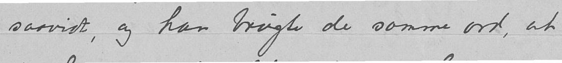saavidt, og han brugte de samme ord, at
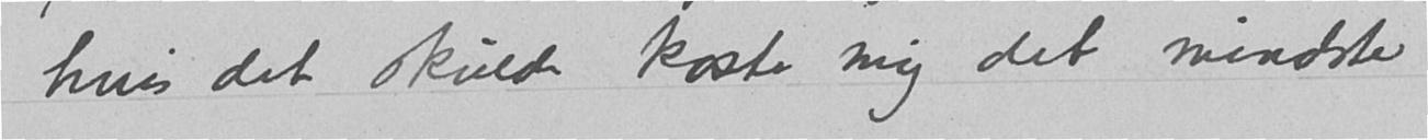- hvis det skulde koste mig det mindste
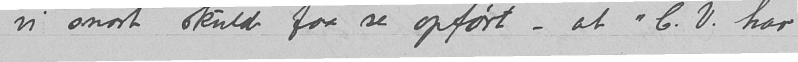vi snart skulde faa se opført – at «C.V. har
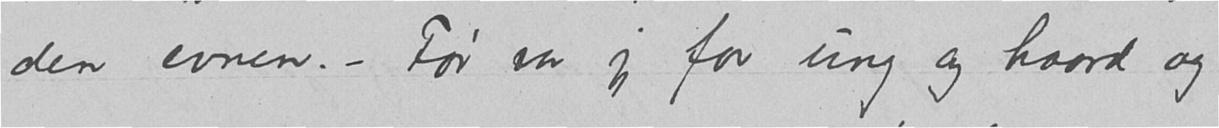den evnen. - Før var jeg for ung og haard og
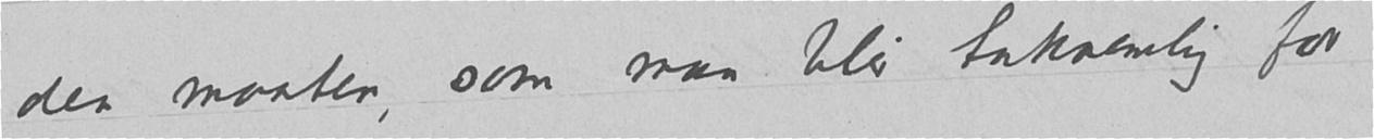den maaten, som man blir taknemlig for". -
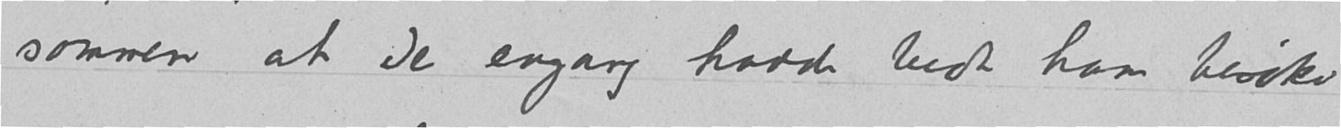sammen at De engang hadde bedt ham besøke
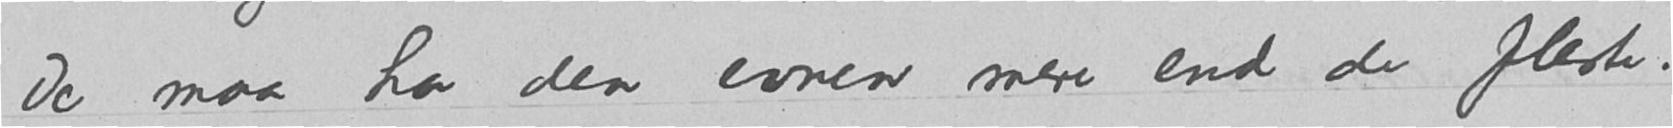De maa ha den evnen mere end de fleste.
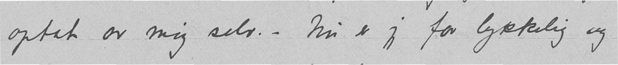optat av mig selv. – Nu er jeg for lykkelig og
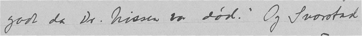godt da Dr. Nissen var død." Og Svarstad
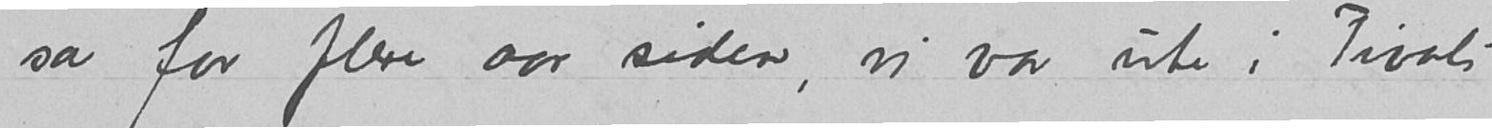sa for flere aar siden, vi var ute i Tivoli
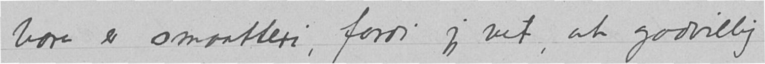bare er smaatteri, fordi jeg vet, at godvillig
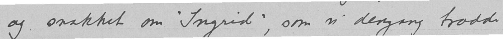og snakket om "Ingrid", som vi dengang trodde
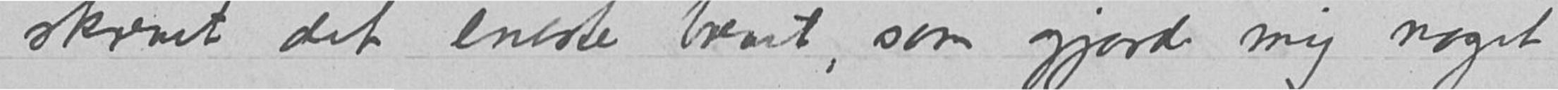skrevet det eneste brevet, som gjorde mig noget
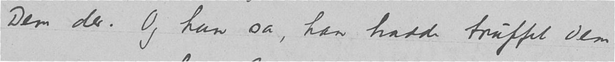Dem der. Og han sa, han hadde truffet Dem
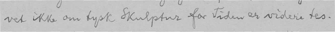vet ikke om tysk Skulptur for Tiden er videre tes.
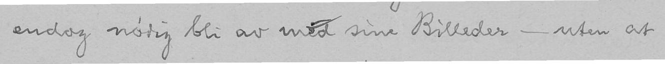endog nødig bli av med sine Billeder - uten at
